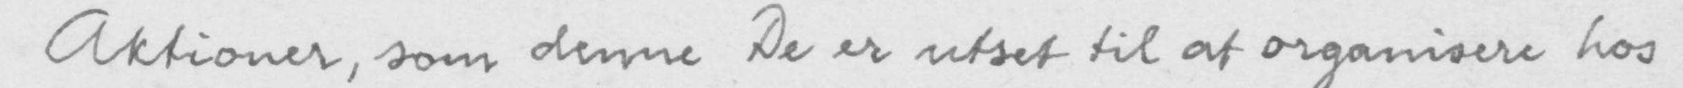Aktioner, som denne De er utset til at organisere hos
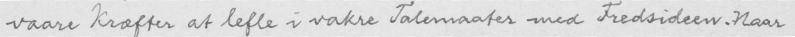vaare Kræfter at lefle i vakre Talemaater med Fredsideen. Naar
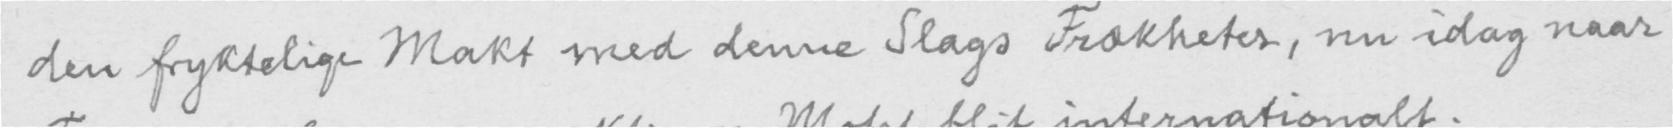den fryktelige Makt med denne Slags Frækheter, nu idag naar
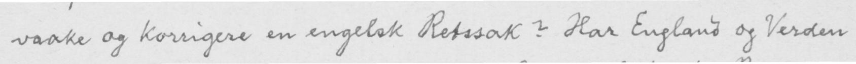vaake og korrigere en engelsk Retssak? Har England og Verden
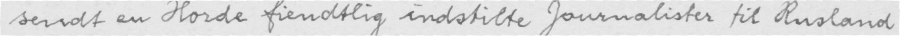sendt en Horde fiendtlig indstilte Journalister til Rusland
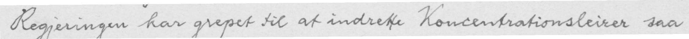Regjeringen har grepet til at indrette Koncentrationsleirer saa
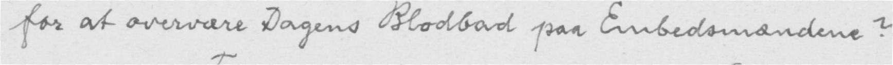for at overvære Dagens Blodbad paa Embedsmændene?
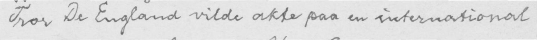Tror De England vilde akte paa en international
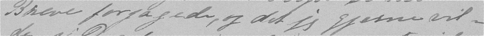Breve forjagede, og det jeg gjerne vil-
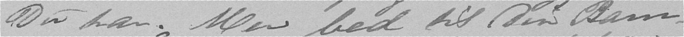Du har. Men bed til din Barn-
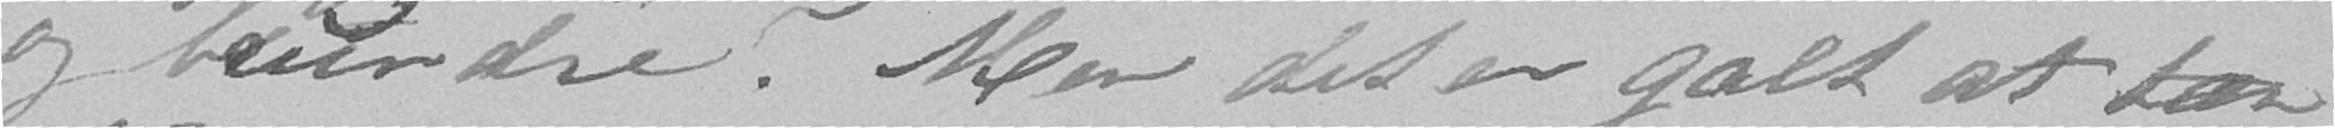og beundre? Men det er galt at tæn-
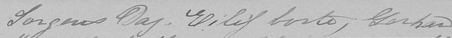Sorgens Dag. Eilif borte, Gerhard
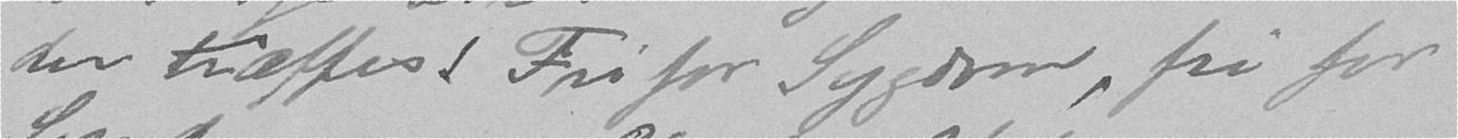der træffes! Fri for Sygdom, fri for
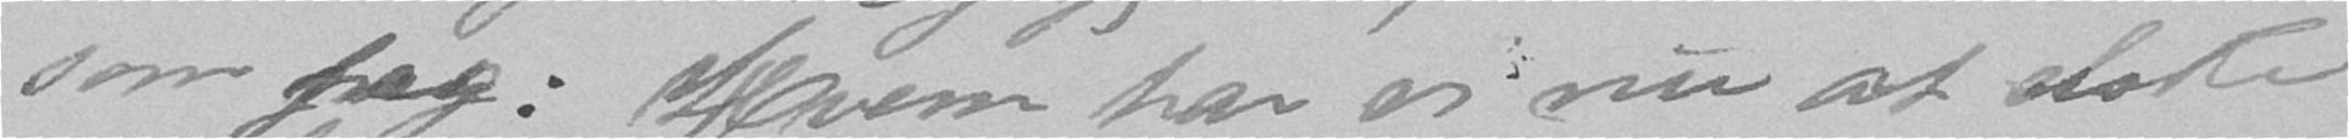som jeg: Hvem har vi nu at elske
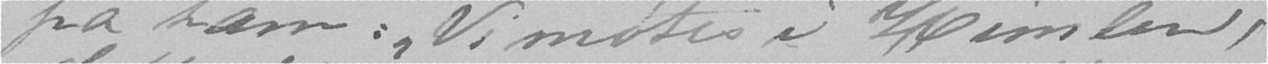fra ham: "Vi møtes i Himlen,
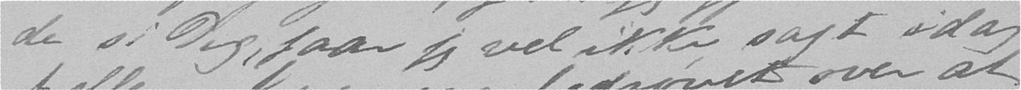de si Dig, faar jeg vel ikke sagt i dag
